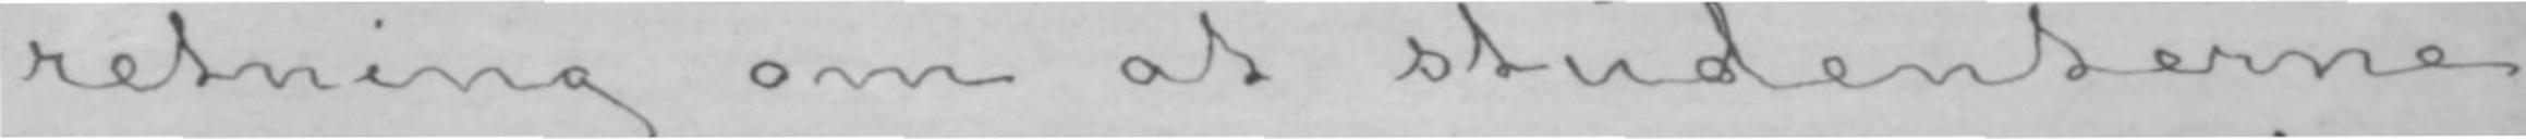retning om at studenterne
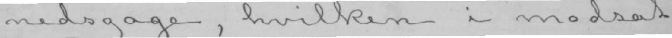nedsgage, hvilken i modsat
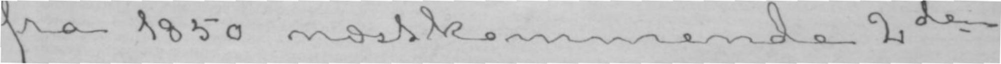fra 1850 næstkommende 2den
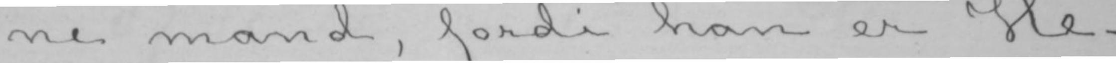ne mand, fordi han er He-
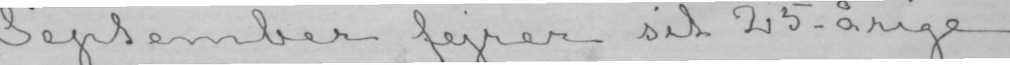September fejrer sit 25-årige
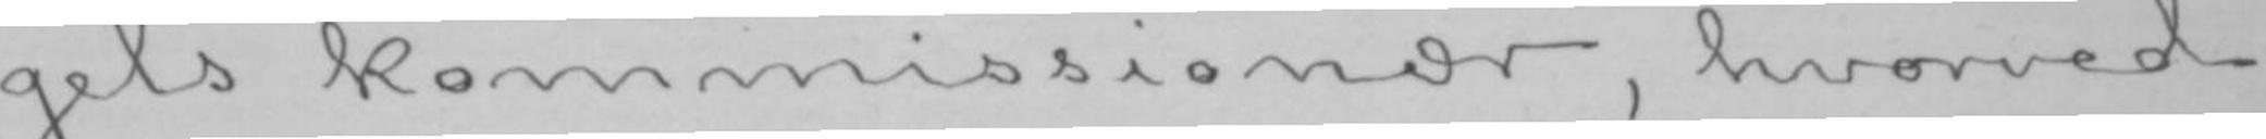gels kommissionær, hvorved
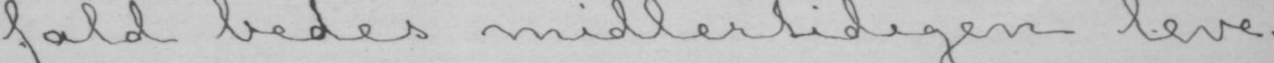fald bedes midlertidigen leve-
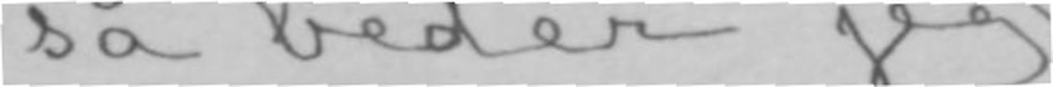så beder jeg
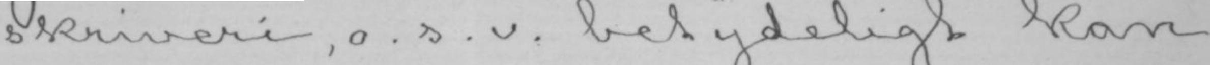skriveri, o. s. v. betydeligt kan
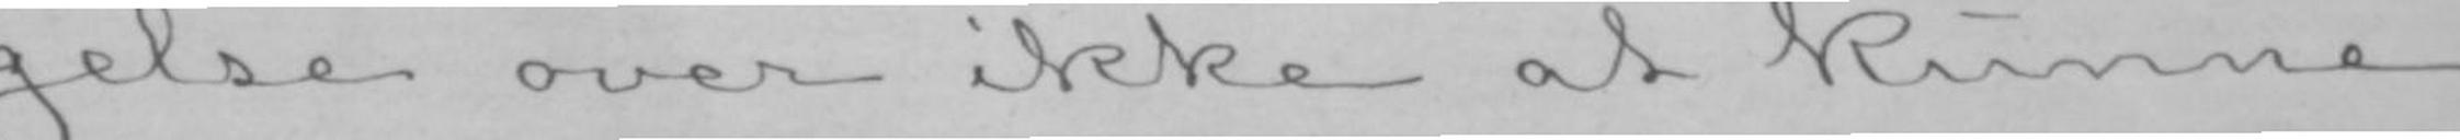gelse over ikke at kunne
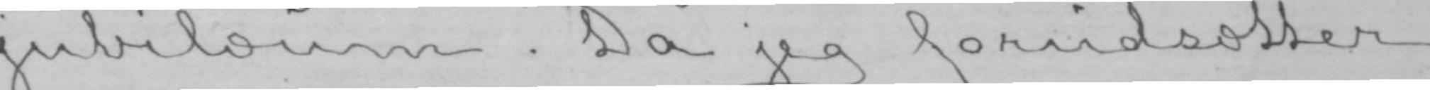jubilæum. Da jeg forudsætter
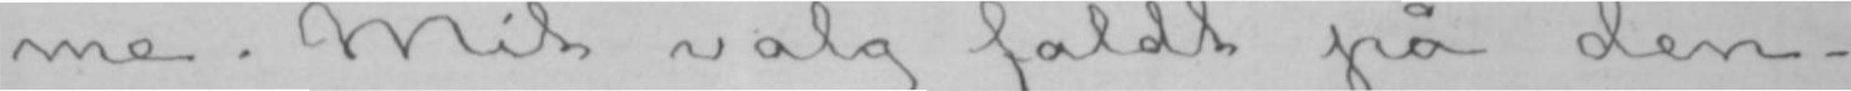me. Mit valg faldt på den-
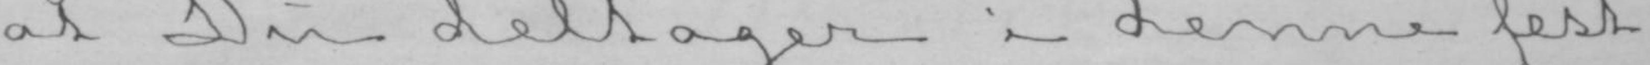at Du deltager i denne fest,
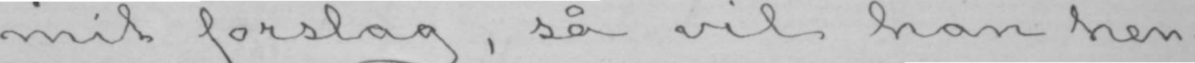mit forslag, så vil han hen-
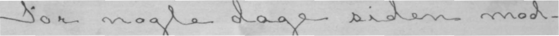For nogle dage siden mod-
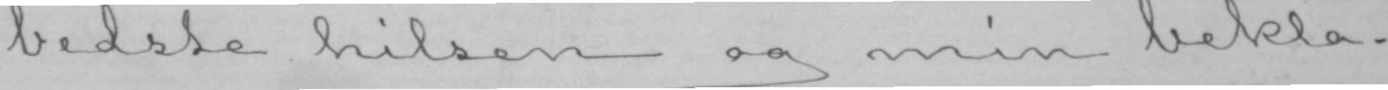bedste hilsen og min bekla-
